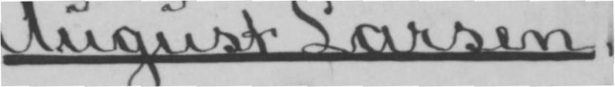August Larsen,
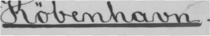København.
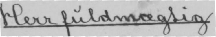Herr fuldmægtig
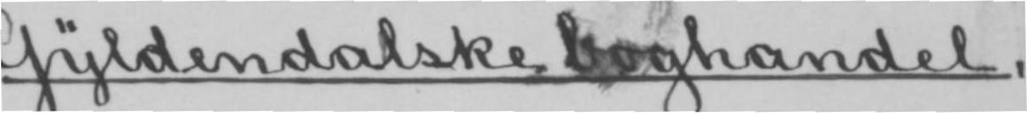Gyldendalske boghandel,
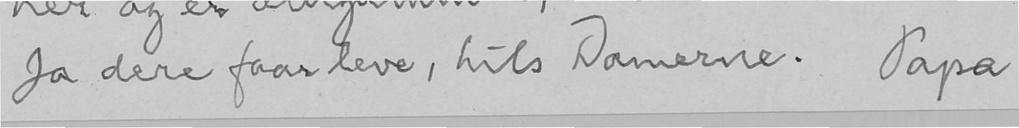Ja dere faar leve, hils Damerne. Papa
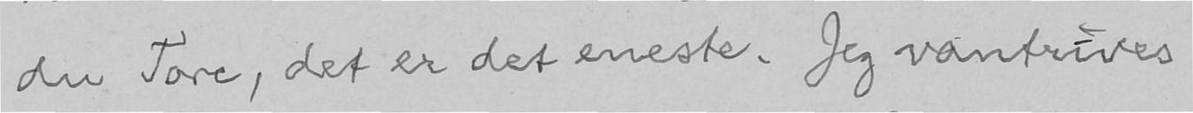du Tore, det er det eneste. Jeg vantrives
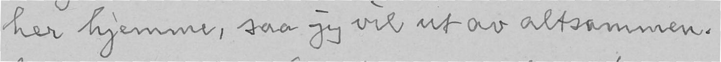her hjemme, saa jeg vil ut av altsammen.
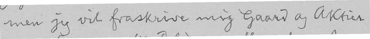men jeg vil fraskrive mig Gaard og Aktier
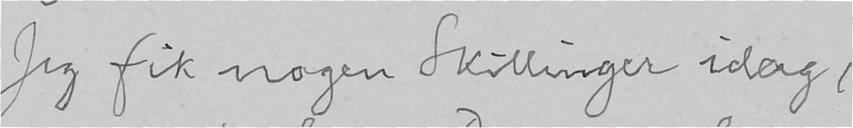Jeg fik nogen Skillinger idag,
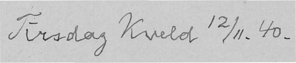Tirsdag Kveld 12/11. 40.
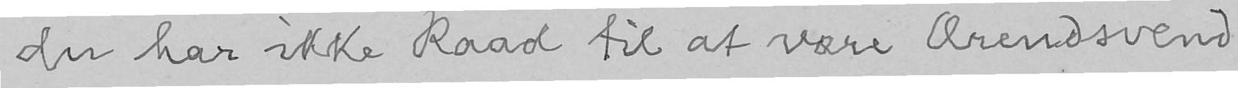du har ikke Raad til at være Ærendsvend
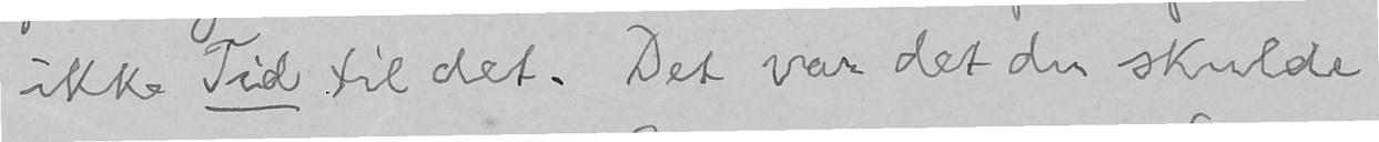ikke Tid til det. Det var det du skulde
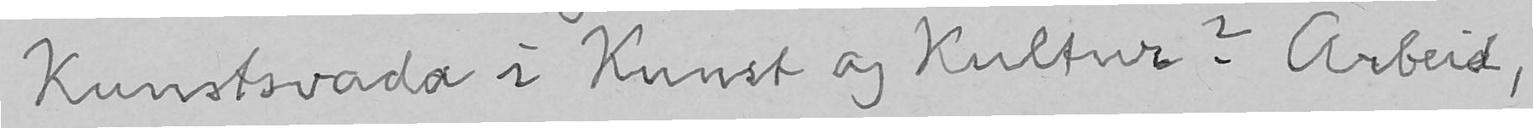Kunstsvada i Kunst og Kultur? Arbeid,
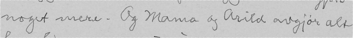noget mere. Og Mama og Arild avgjør alt
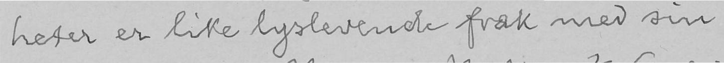heter er like lyslevende fræk med sin
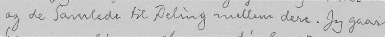og de Samlede til Deling mellem dere. Jeg gaar
